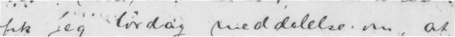fik jeg lørdag meddelelse om, at
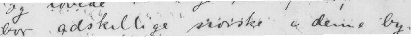bor adskillig norske i denne by-
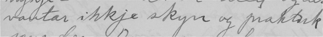vantar ikkje skyn og praktisk
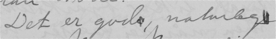Det er god, naturleg
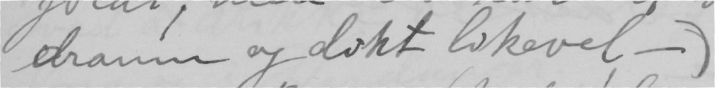dramm og dikt likevel –
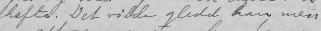hefte. Det vilde gledd han meir
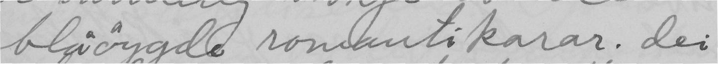blåöygde romantikarar, dei
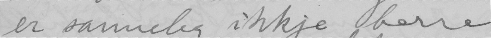er sanneleg ikkje berre
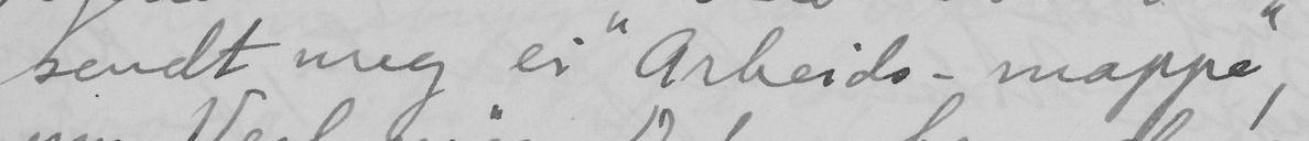sendt meg ei "arbeids-mappe",
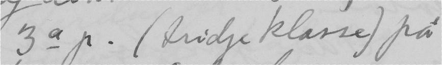3a p. (tridje klasse) på
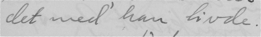det med han livde.
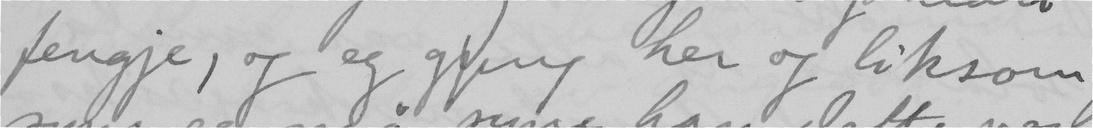fengje, og eg gjeng her og liksom
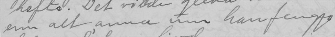enn alt anna um han fengjo
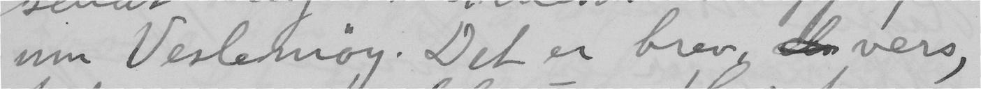um Veslemöy. Det er brev,  vers,
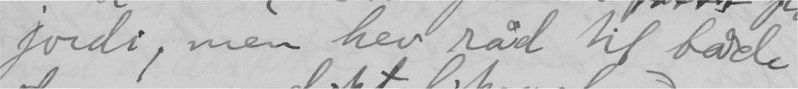jordi, men hev råd til både
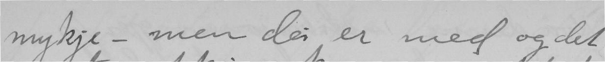mykje – men dei er med og det
